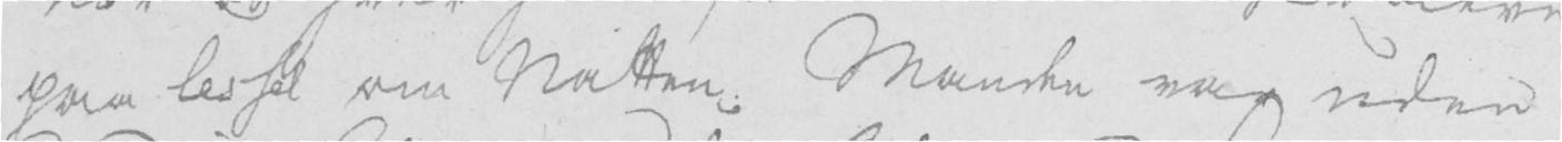paa lesset om Natten; Manden var uden
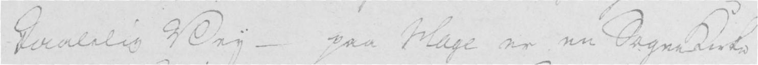taalelig Vey - Paa Mage er en Sogne Kirke
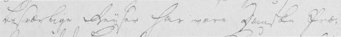besværlige Reyser har vore Danske Præ-
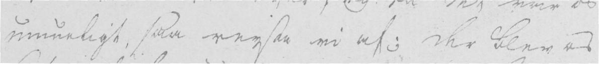umueligt, saa reyste vi af; der blev os
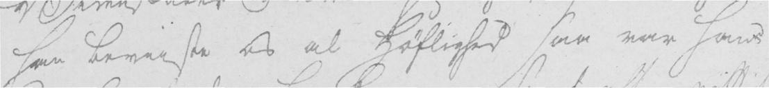han beviiste os al Høflighed saa var hands
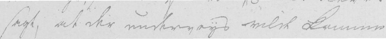sagt, at der underveys vilde komme
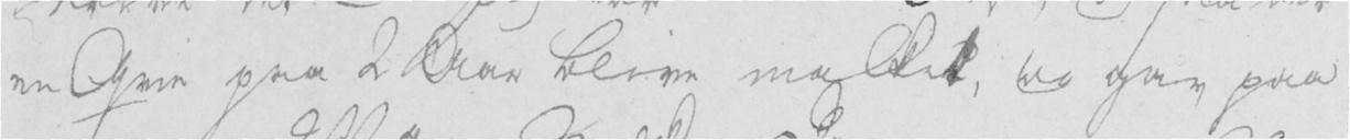en Qvi paa 2 Aar blive melket, og gav paa
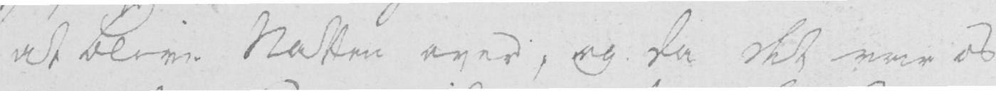at blive Natten over, og da det var os
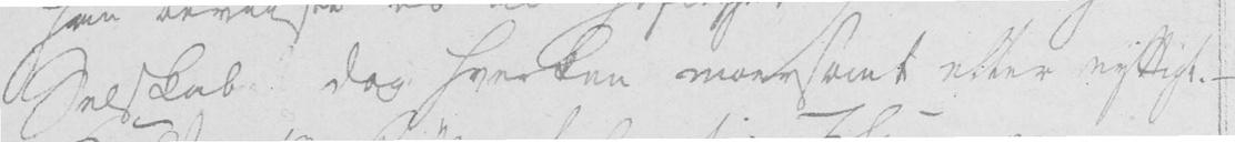Selskab Dog hverken maarsomt eller nyttigt. -
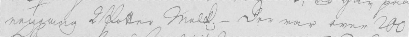eengang 2 Potter Melk; - Der var over 200
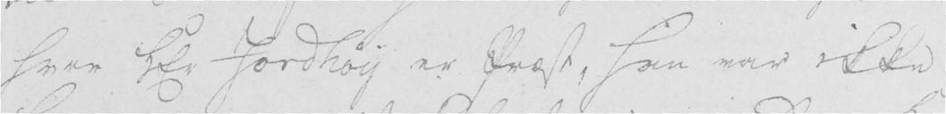hvor Hr Jordhøy er Præst, han var ikke
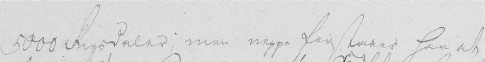5000 Rigsdaler; men neppe forstaaer han at
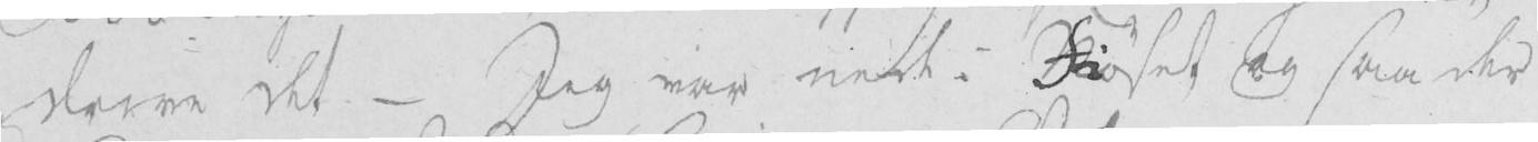drive det. - Jeg var ned i Fiøset og saa der
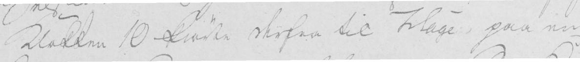Klokken 10 kiørte derfra til Mage, paa en
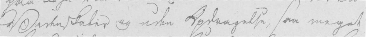Videnskaber og uden Opdragelse, saa meget
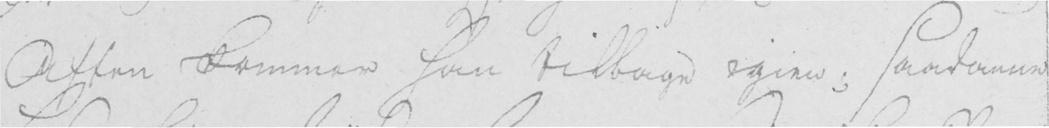Aften kommer han tilbage igien; saadanne
